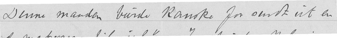Denne manden burde kanske faa sendt ut en
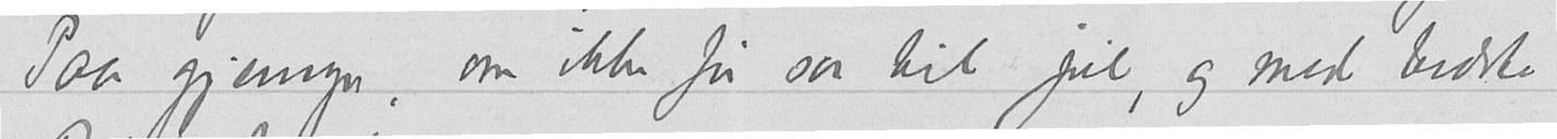Paa gjensyn, om ikke før saa til jul, og med bedste
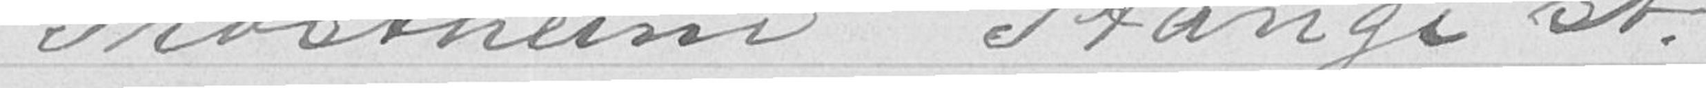Trøstheim Stange st.
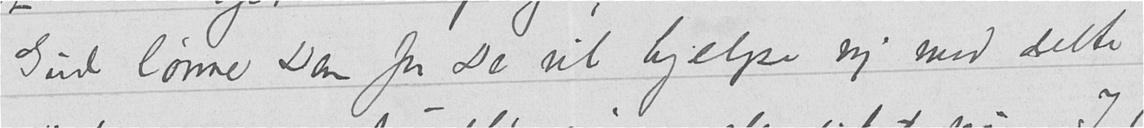Gud lønne Dem for De vil hjelpe mig med dette
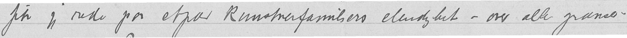fik jeg rede paa etpar kunstnerfamiliers elendighet – over alle grænser.
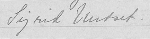Sigrid Undset.
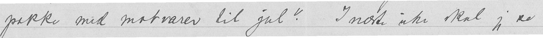pakke med matvarer til jul? I næste uke skal jeg se
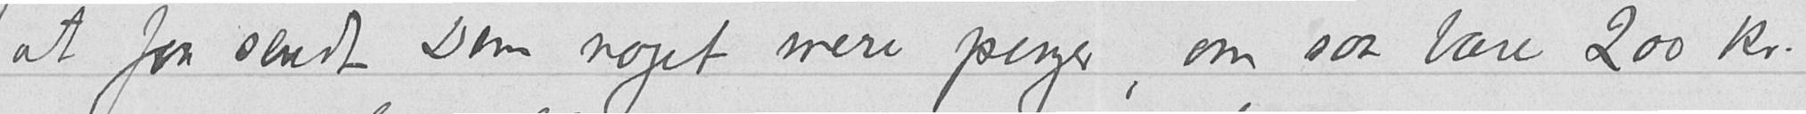at faa sendt Dem noget mere penger, om saa bare 200 kr.
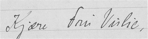Kjære Fru Vislie,
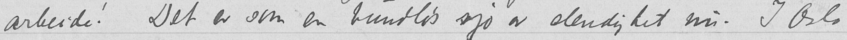arbeide! Det er som en bundløs sjø av elendighet nu. I Oslo
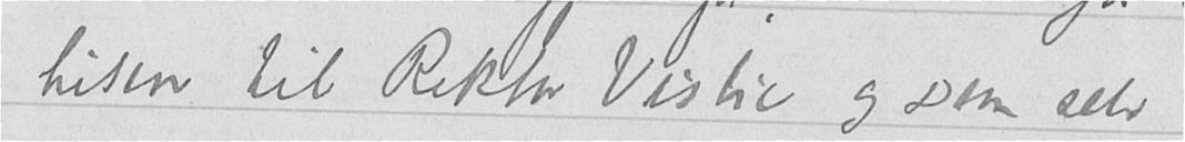hilsen til Rektor Vislie og Dem selv
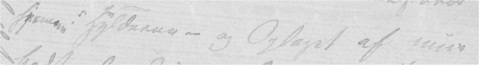hjemme i Hylderne – og Oplaget af min
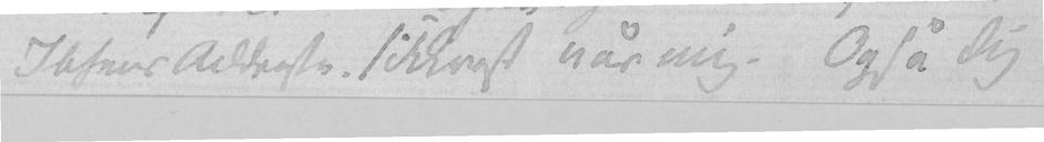Ibsens Addresse, sikkrest når mig. Også Dig
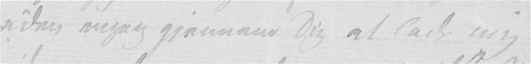uden engang gjennem Dig at lade mig
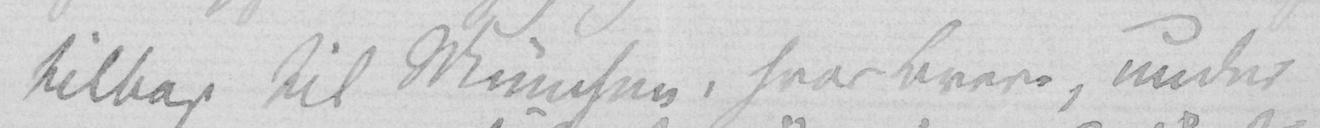tilbage til München, hvor Breve, under
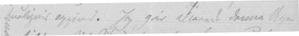turligvis opgives. Jeg går allerede denne Uge
